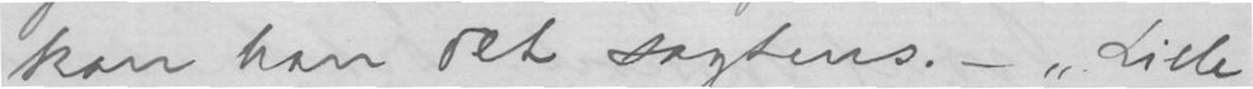kan han det sagtens.- "Lille
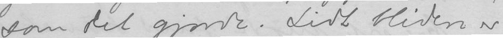som det gjorde. Lidt bliden er
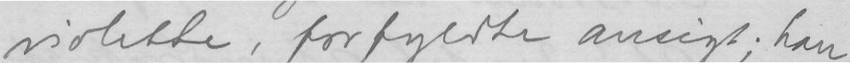violette, forfyldte ansigt; han
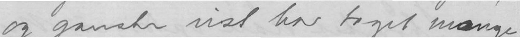og ganske vist har taget mange
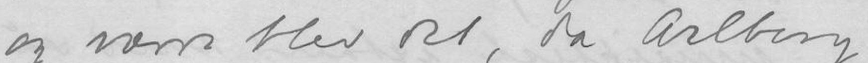og værre blev det, da Arlberg
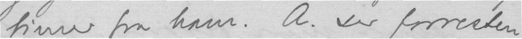timer fra ham. A. ser forresten
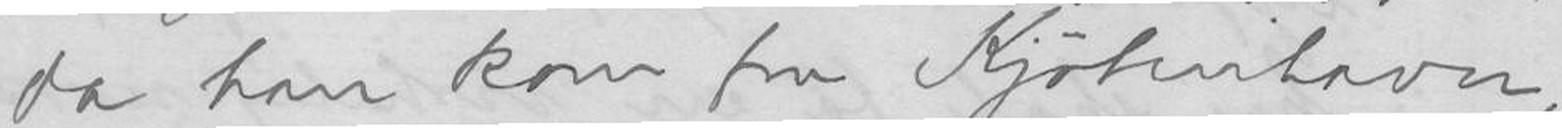da han kom fra Kjøbenhavn,
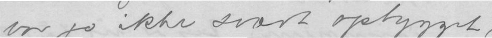var jo ikke svært opbygget,
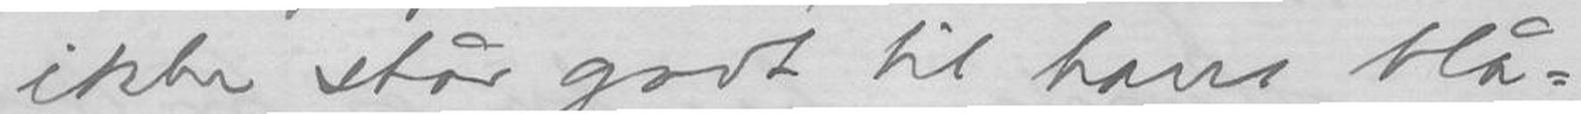ikke står godt til hans blå-
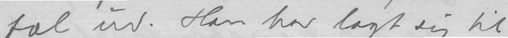fæl ud. Han har lagt sig til
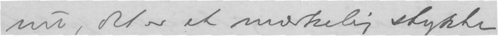nu, det er et mærkelig stykke
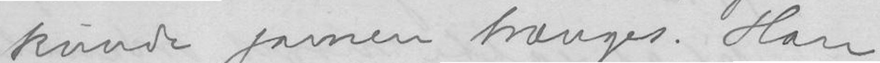kunde jamen trænges. Han
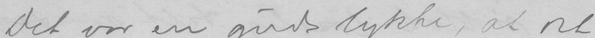Det var en guds lykke, at det
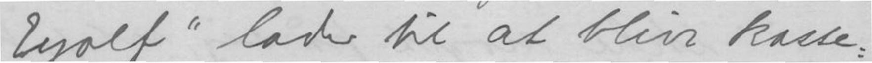Eyolf" lader til at blive kasse-
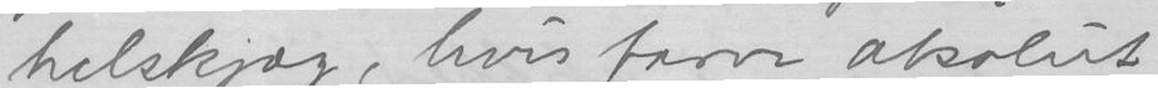helskjæg, hvis form absolut
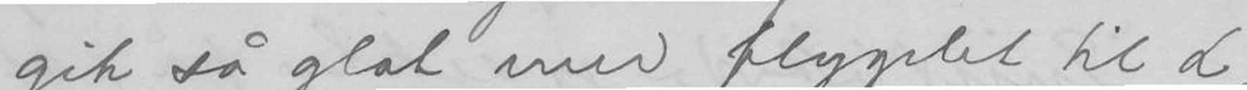gik så glat med flygelet til L,
